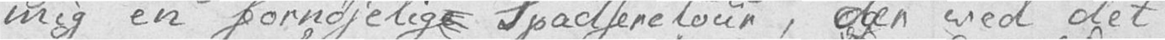mig en fornøjelige Spadseretour, der ved det
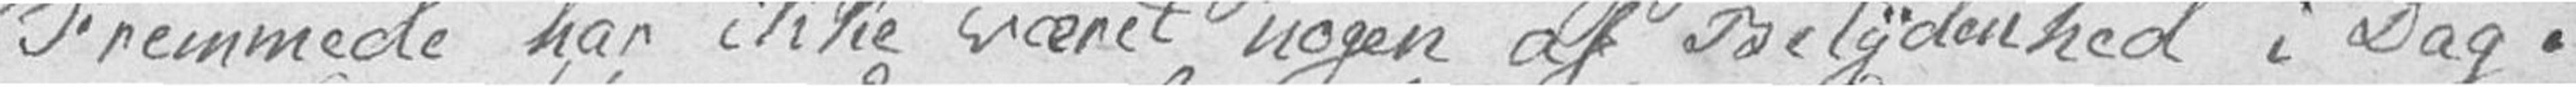Fremmede har ikke været nogen af Betydenhed i Dag.
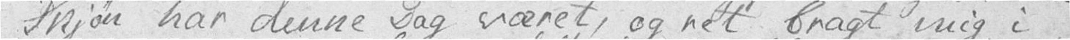Skjøn har denne Dag været, og ret bragt mig i
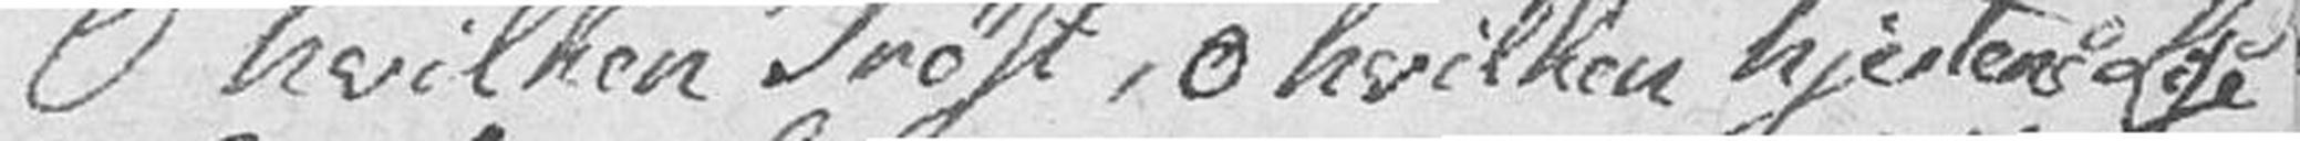O hvilken Trøst, o hvilken hjertens Lise
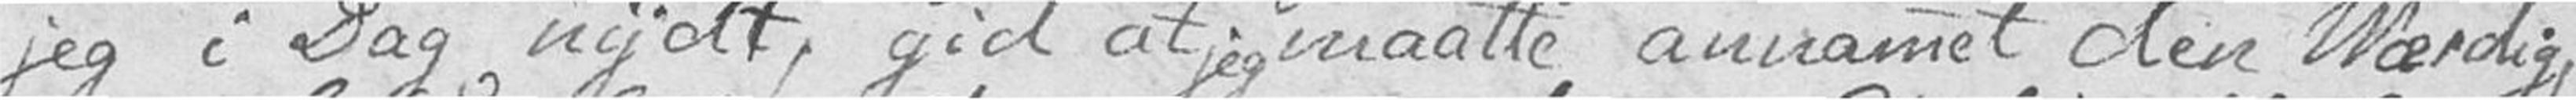jeg i Dag nydt, gid at jeg maatte annammet den Værdig,
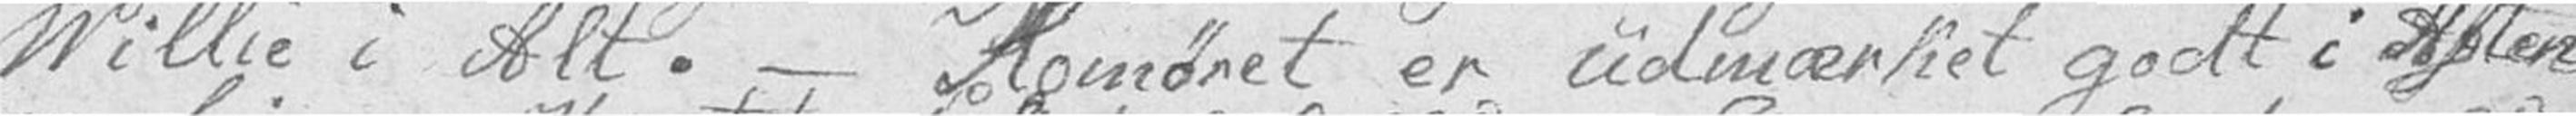Villie i Alt. – Homøret er udmærket godt i Aften
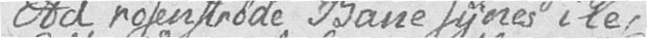Ad rosenstrøde Bane synes i le,
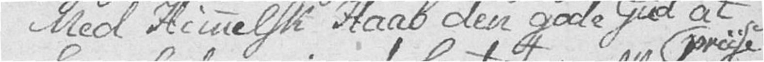Med Himmelsk Haab den gode Gud at priise
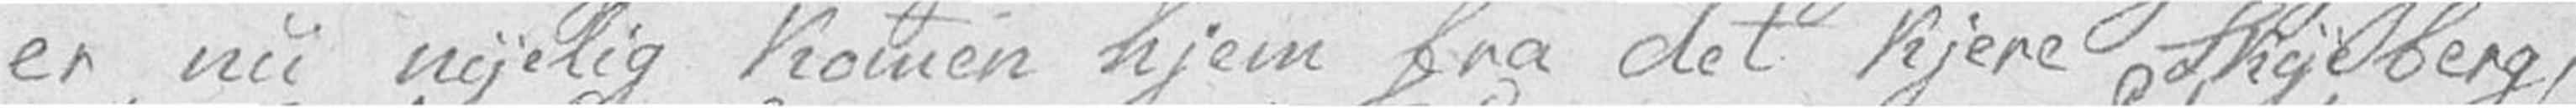er nu nyelig kommen hjem fra det kjere Skyeberg,
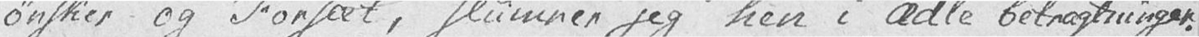ønsker og Forsæt, slumrer jeg hen i ædle betragtninger.
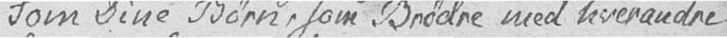Som Dine Børn, som Brødre med hverandre
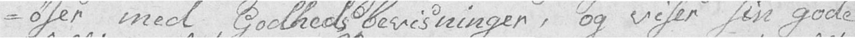-øser med Godhedsbevisninger, og viser sin gode
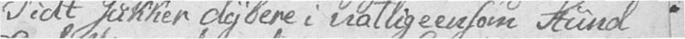Tidt sukker dybere i natlig eensom Stund
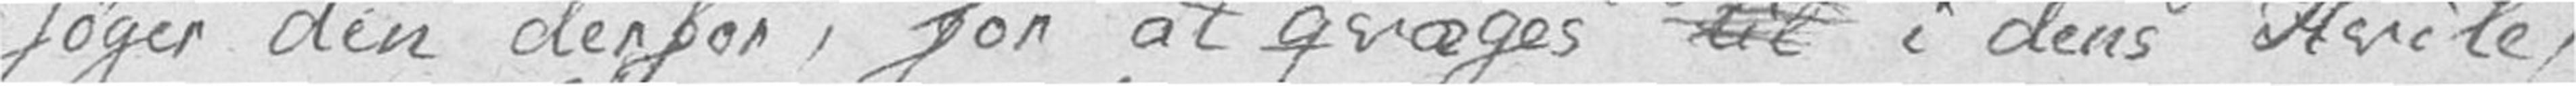søger den derfor, for at qvæges til i dens Hvile,
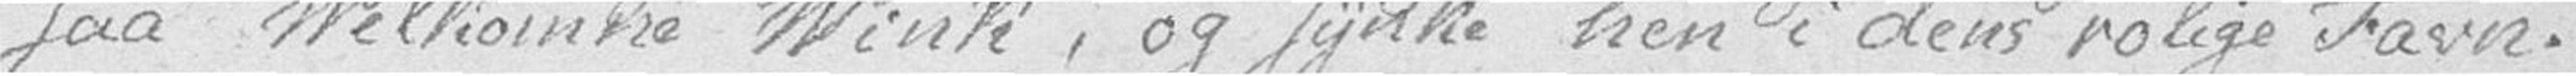saa Velkomne Vink, og synke hen i dens rolige Favn.
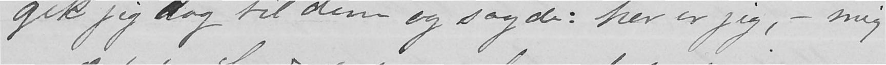gik jeg dog til dem og sagde: her er jeg, - mig
